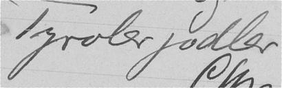Tyrolerjodler
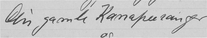Din gamle Kanapeesanger
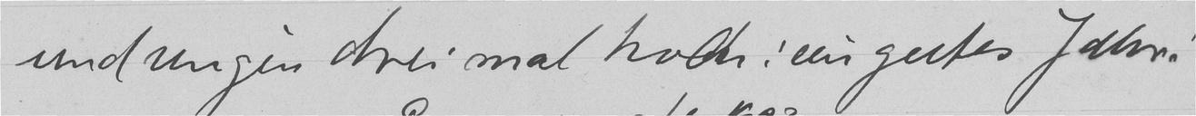und unzer drei mal haben: ein gutes Jahr!
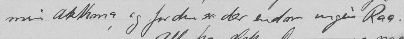min Asthma, og for den er der endnu ingen Raa.
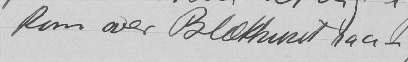kom over Blækhuset saa -
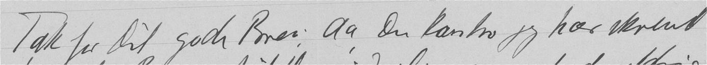Tak for dit gode Brev; Aa, du kan tro jeg har skrevet
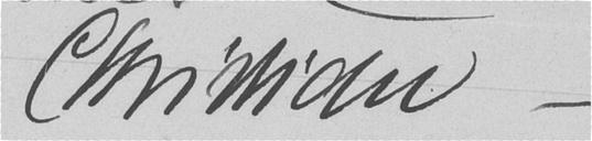Christian -
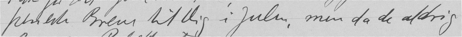peneste Brev til dig i Julen, men da de aldrig
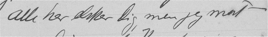Alle her elsker dig, men jeg mest -
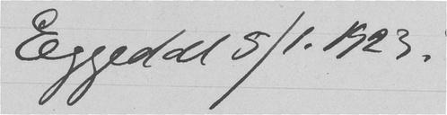Eggedal 5/1. 1923.
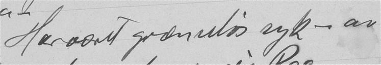Har været grænseløs syk - av
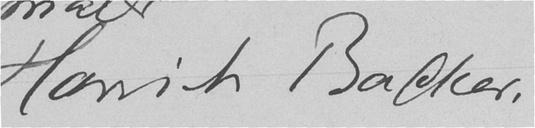Harriet Backer.
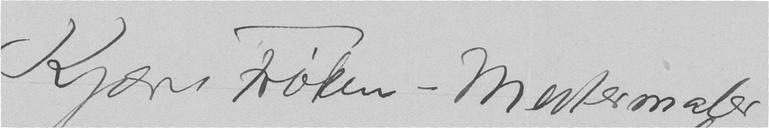Kjære Frøken-Mestermaler
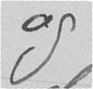og
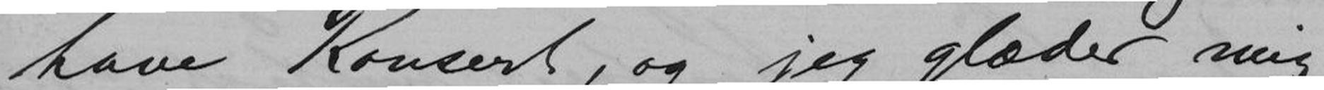have Konsert, og jeg glæder mig
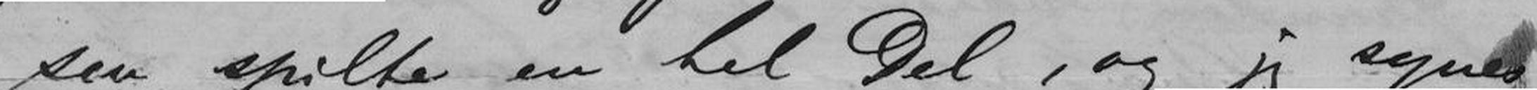sen spilte en hel Del, og jeg synes
